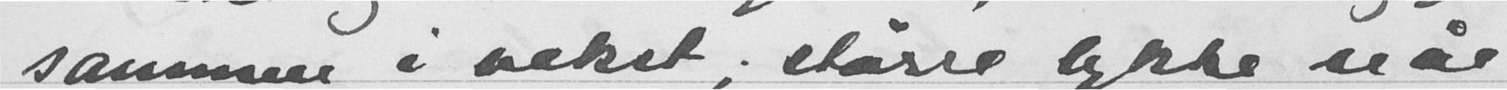sammen i vekst; større lykke når
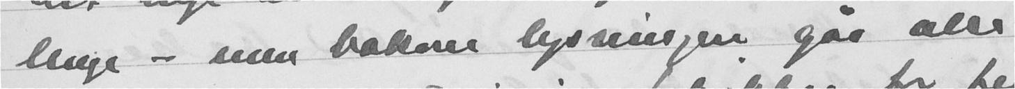lenge - men bakom lysningen går alle
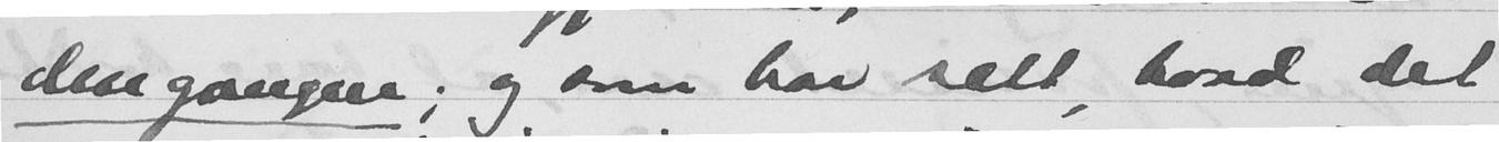den gangen; og som har sett hvad det
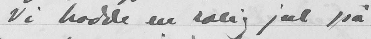Vi hadde en rolig jul på
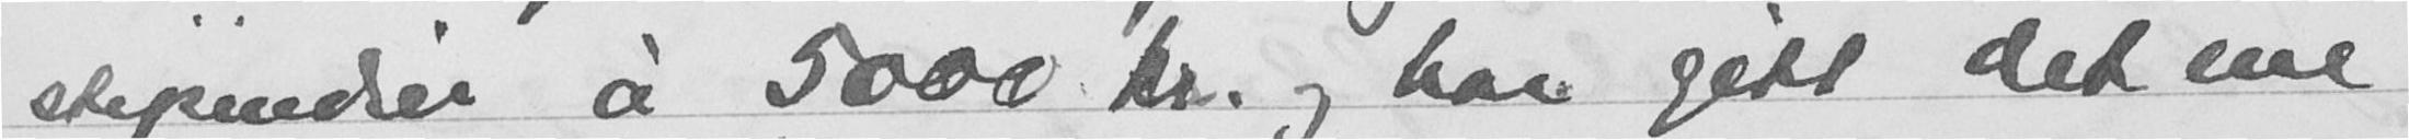stipender à 5000 kr. og har gitt det ene
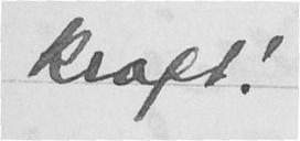kraft.
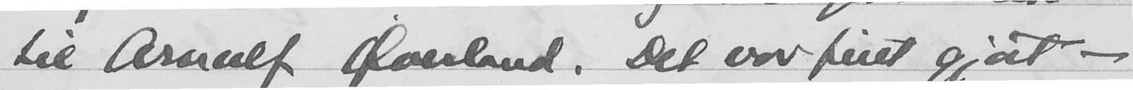til Arnulf Øverland. Det var fint gjort -
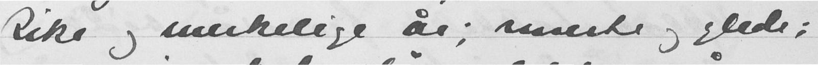Rike og merkelige år; rmante og glede;
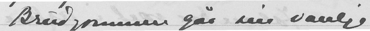Brudgommen går sin vanlige
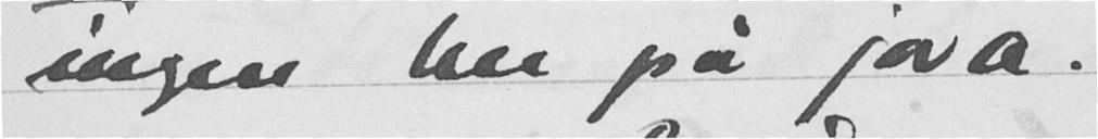ingen, her på jora.
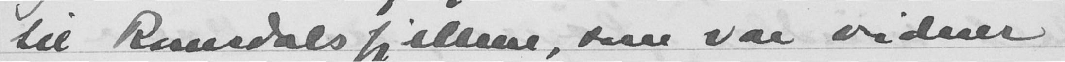til Romsdalsfjellene, som var videre
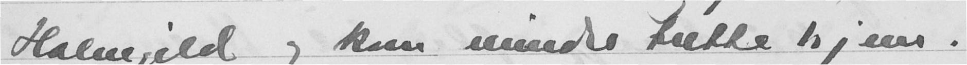Holmgild og kom mindre fulle hjem.
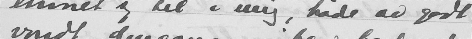emnet sig til i mig, både av godt
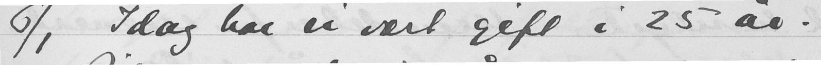6/1 Idag har vi vært gift i 25 år.
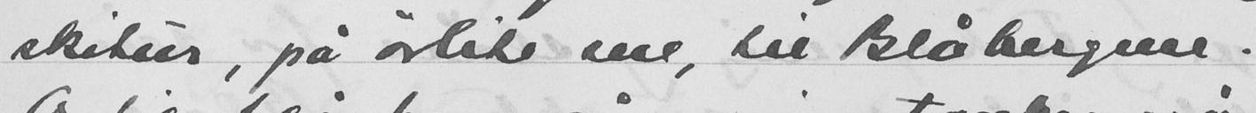skitur, på ørlite sne, til Blåbergene.
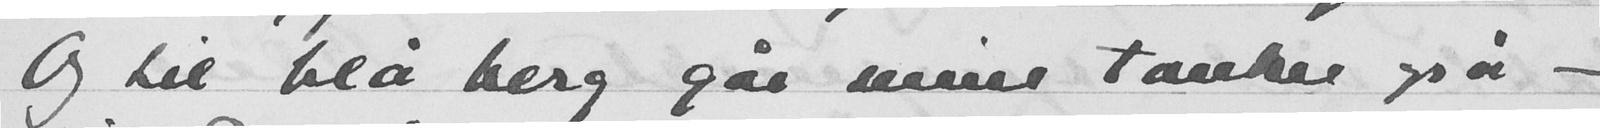Og til blå berg går mine tanker også -
